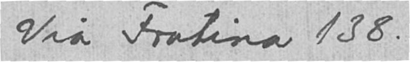Via Fratina 138.
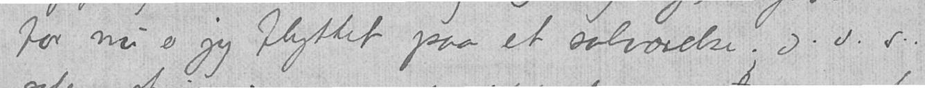for nu er jeg flyttet paa et solværelse. D.v.s.
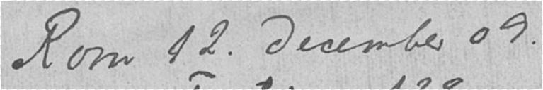Rom 12. December 09.
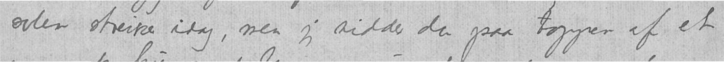solen streiker idag, men jeg sidder da paa toppen af et
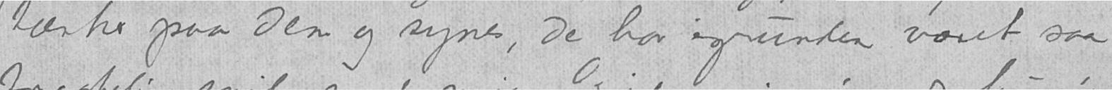tænker paa Dem og synes, De har igrunden været saa
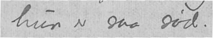hun er saa sød.
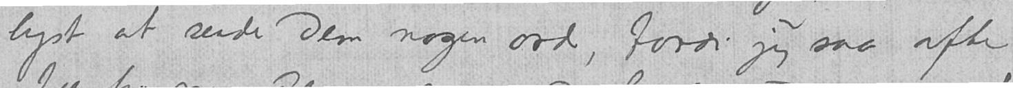lyst at sende Dem nogen ord, fordi jeg saa ofte
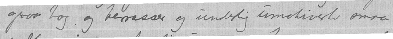graa tag og terrasser og underlig umotiverte smaa
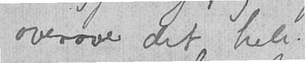overover det hele.
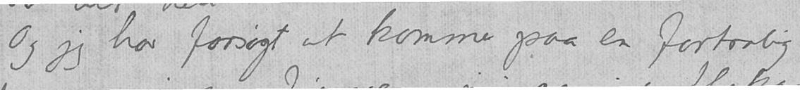Og jeg har forsøgt at komme paa en fortrolig
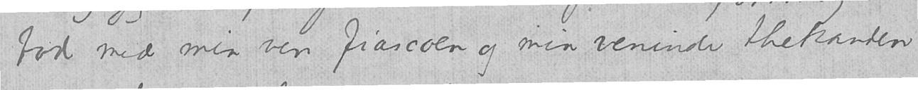fod med min ven fiascoen og min veninde thekanden
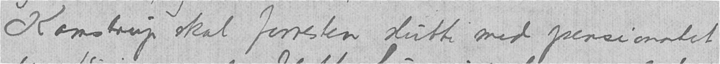Kamstrup skal forresten slutte med pensionatet
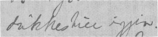dukkestue igjen.
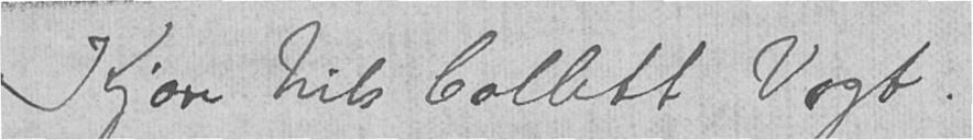Kjære Nils Collett Vogt!
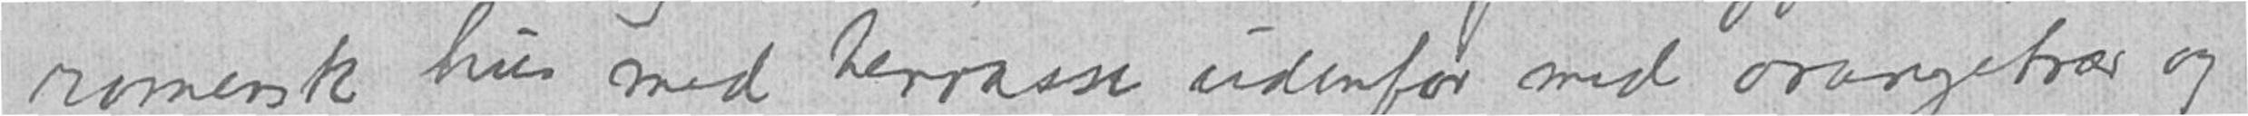romersk hus med terrasse udenfor med orangetrær og
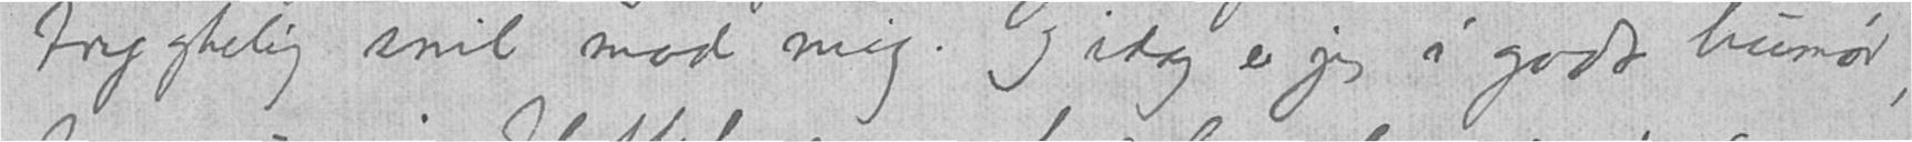frygtelig snil mod mig. Og idag er jeg i godt humør,
